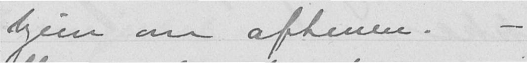hjem om aftenen. -
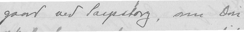gaard ved Sarpsborg, som Don
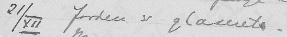21/XII Jorden er glaserete.
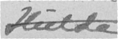Hulda
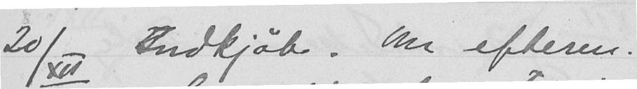20/XII Indkjøb. Om efterm.
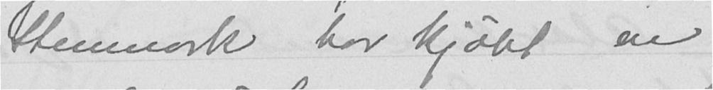Stenmark har kjøbt en
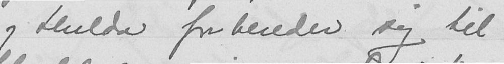og Hulda forbereder sig til
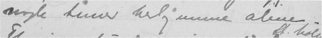nogle timer herhjemme alene.
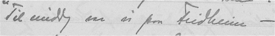Til middag var vi paa Fridheim -
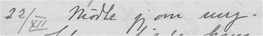22/XII Mødte jeg om mrg.
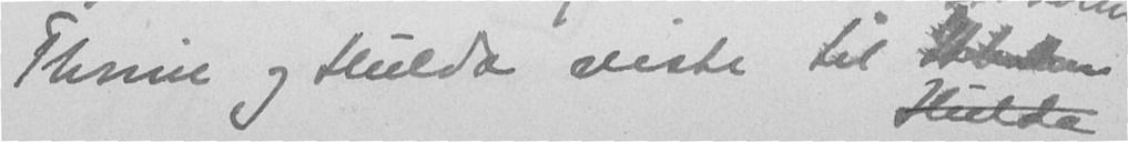Thrine og Hulda reiste til Stholm
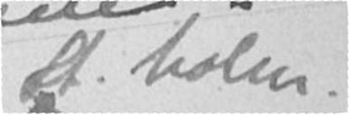St. holm.
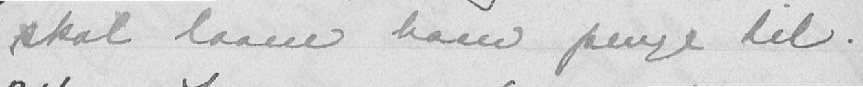skal laane ham penge til.
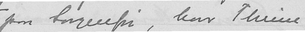paa Sorgenfri, hvor Thrine
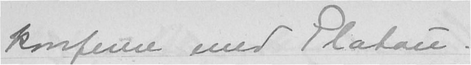konferere med Platou.
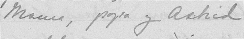Mama, papa og Astrid
